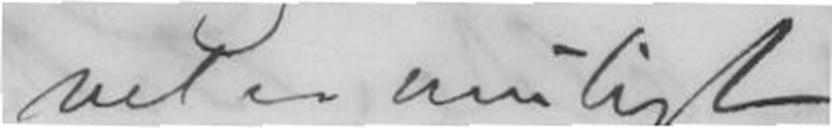vel er muligt
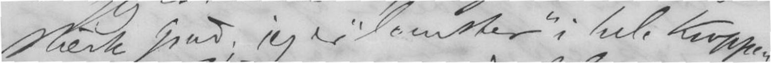stærk Grad; jeg er "læmster" i hele Kroppen
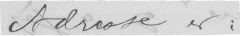Adresse er:
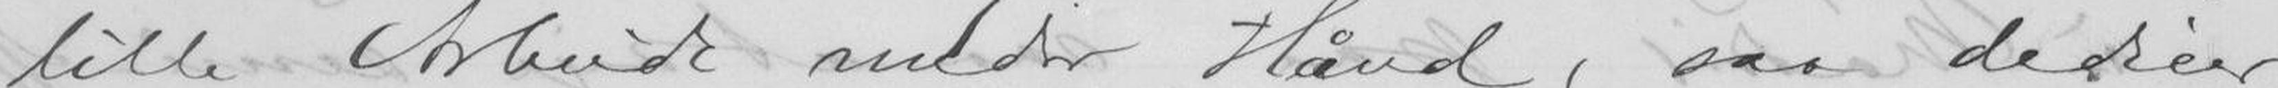lille Arbeide under Hånd, saa dedicer
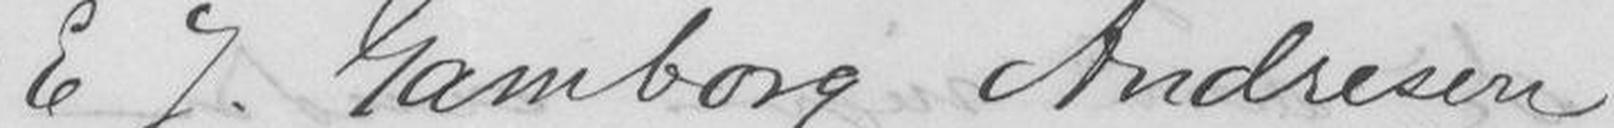E J. Gamborg Andresen
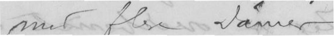med flere Damer.
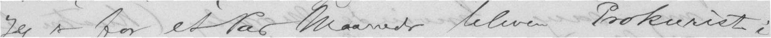Jeg er for et Par Maaneder bleven Prokurist i
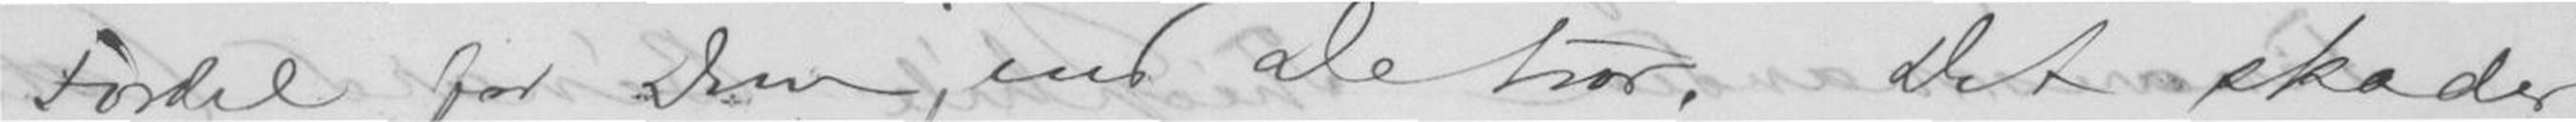Fordel for Dem, end De tror. Det skader
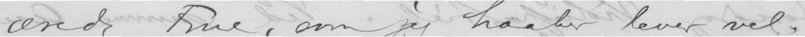ærede Frue, som jeg haaber lever vel. -
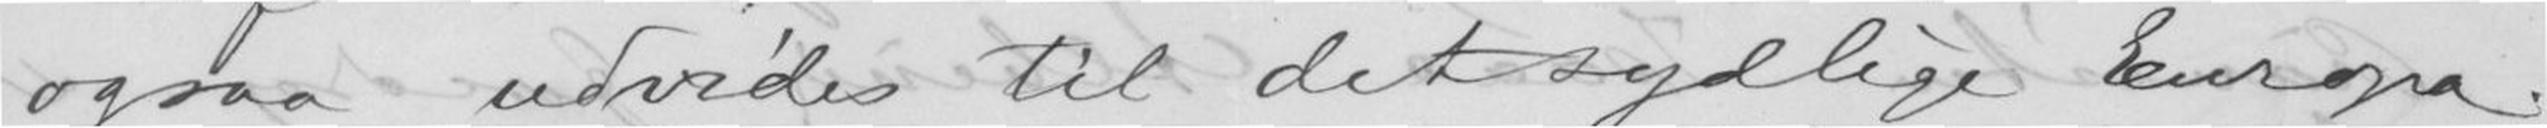ogsaa udvides til det sydlige Europa.
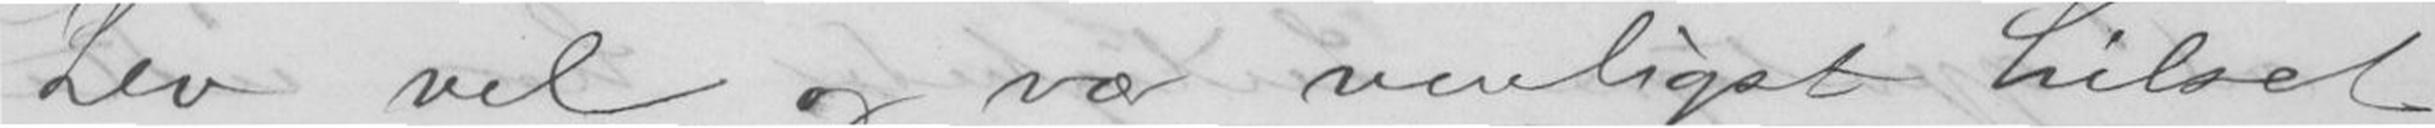Lev vel og vær venligst hilset
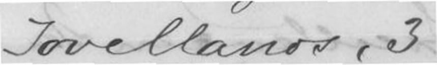Jovellanos, 3
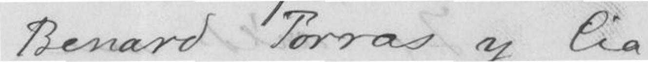Bernard Torras y Cia
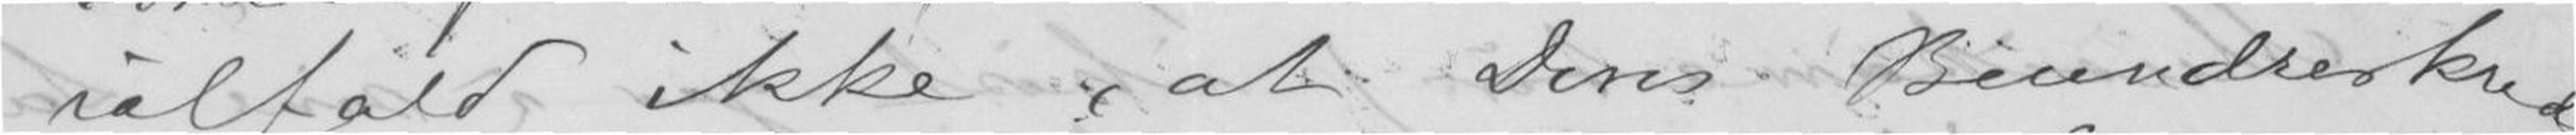ialfald ikke, at Deres Beundrerkreds
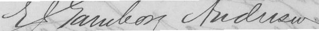E J Gamborg Andresen
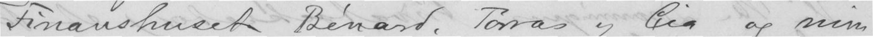Finanshuset Bénard. Torras y Cia og min
